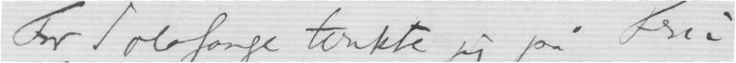For Solosange tenkte jeg på Fru
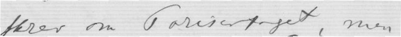skrev om Parisertoget, men
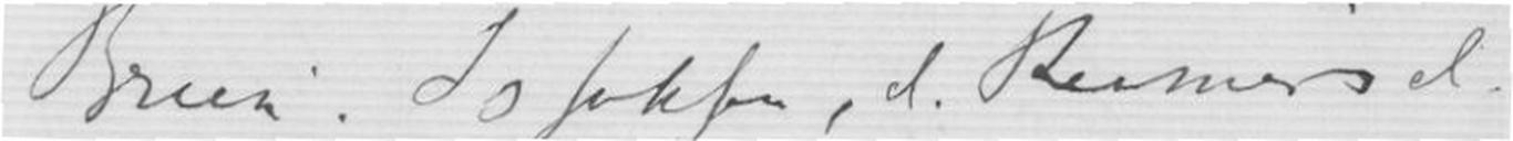Brun. Issaksen, el. Reimers el.
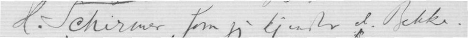H. Schirmer, som jeg kjender el. Bakke-
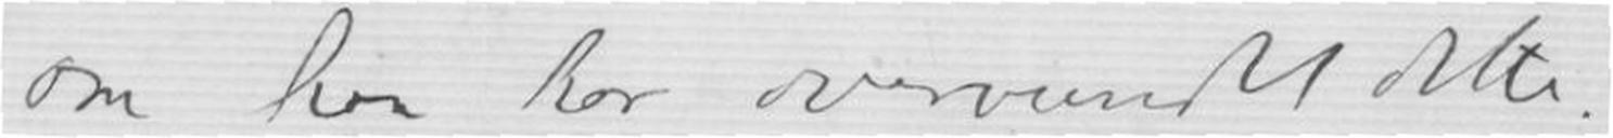om han har overvundet dette.
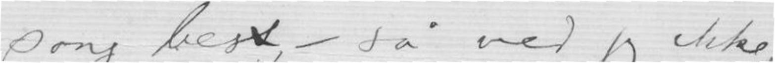sang best, - så ved jeg ikke,
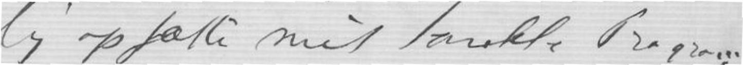lig opsætte mit tænkte Program.
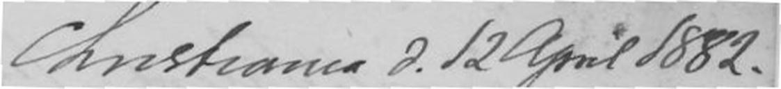Christiania d. 12 April 1882.
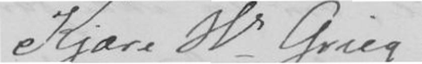Kjære Hr Grieg.
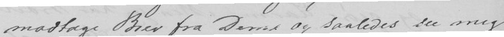modtage Brev fra dem og saaledes se mig
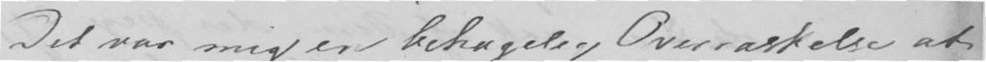Det var mig en behagelig Overraskelse at
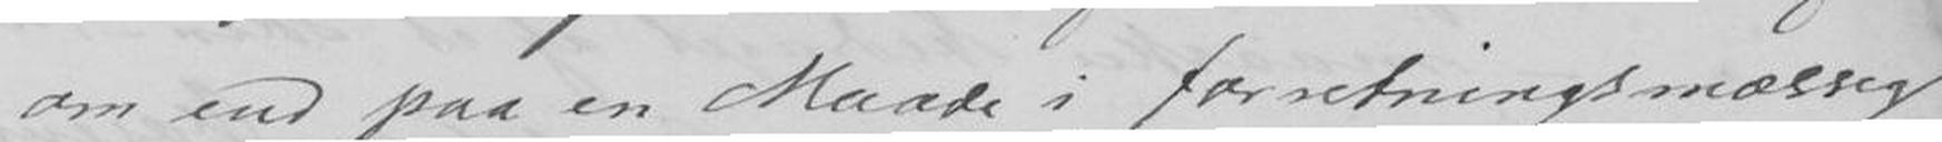- om end paa en Maade i forretningsmæssig
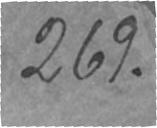269.
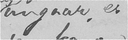angaar, er
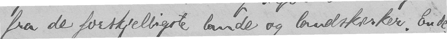fra de forskjelligste lande og landskirker. Ende-
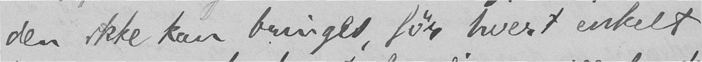den ikke kan bringes før hvert enkelt
